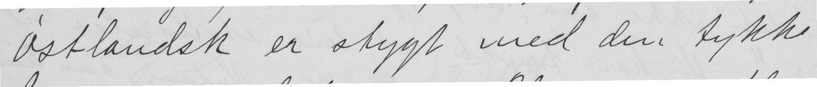Østlandsk er stygt med den tykke
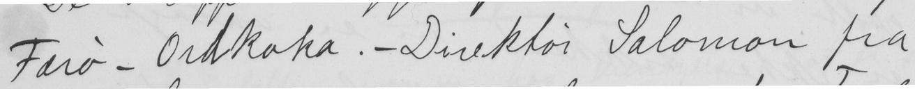Færø - Ordboka. - Dirktør Salomon fra
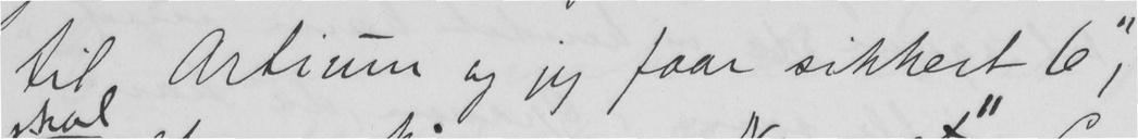til Artium og jeg faar sikkert 6,"
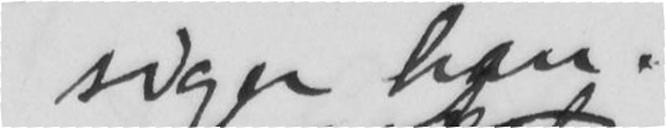siger han.
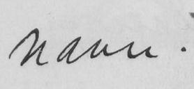navn.
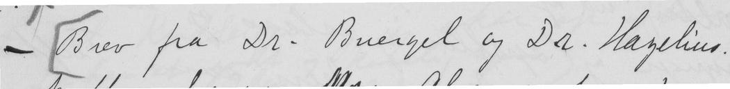- Brev fra Dr. Buergel og Dr. Hagelius.
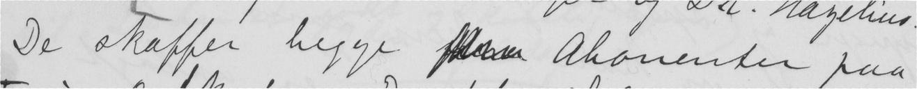De skaffer begge  Abonenter paa
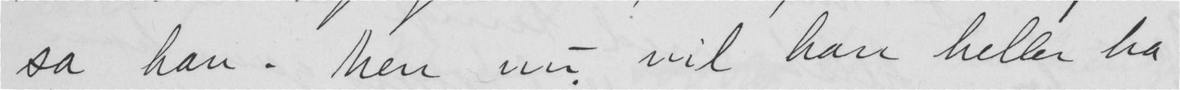sa han. Men nu vil han heller ha
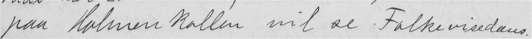paa Holmenkollen vil se Folkevisedans.
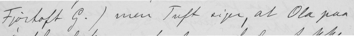Fjørtoft G.) men Tuft siger, at Ola paa
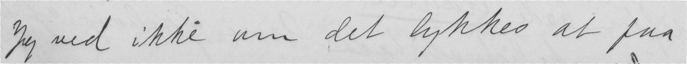Jeg ved ikke om det lykkes at faa
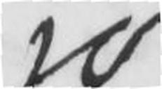jo
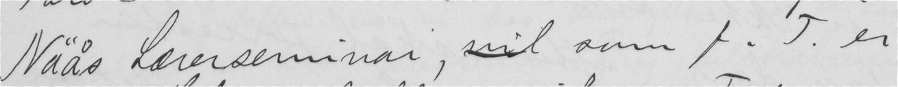Näås Lærerseminar,  som f. T. er
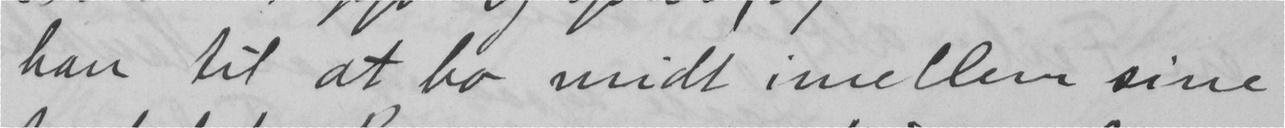han til at ho midt imellem sine
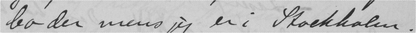bo der mens jeg er i Stockholm.
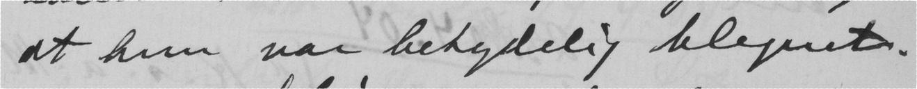at hun var betydelig blegnet.
